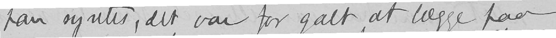han syntes, det var for galt at lægge paa
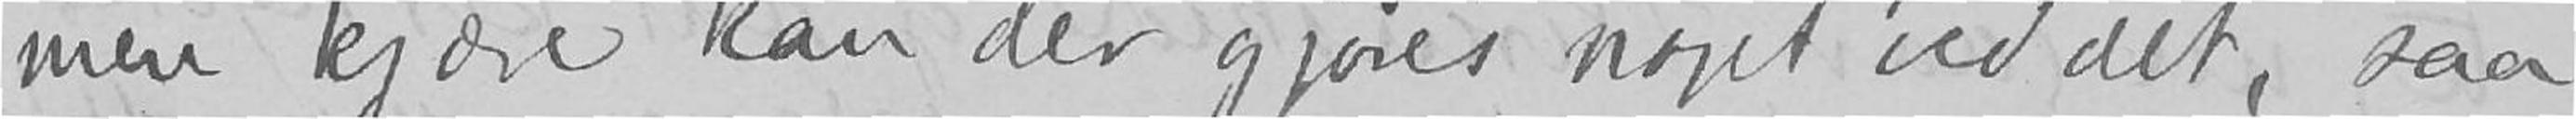men kjære kan der gjøres noget ved det, saa
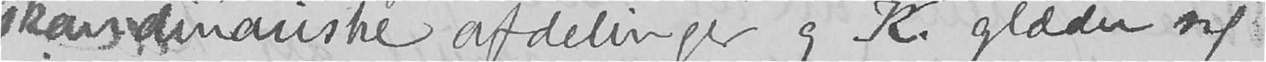skandinaviske afdelinger og K. glæder sig
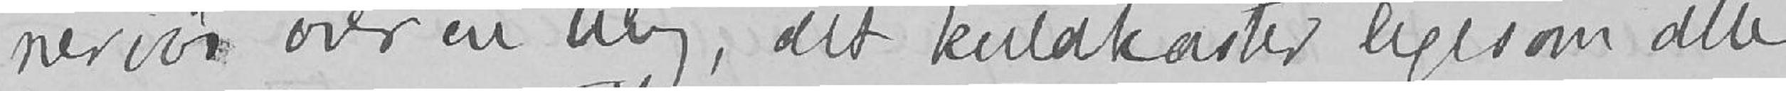nervøs over en ting, det kuldkaster ligesom alle
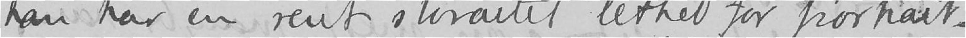han har en rent storartet lethed for portrait-
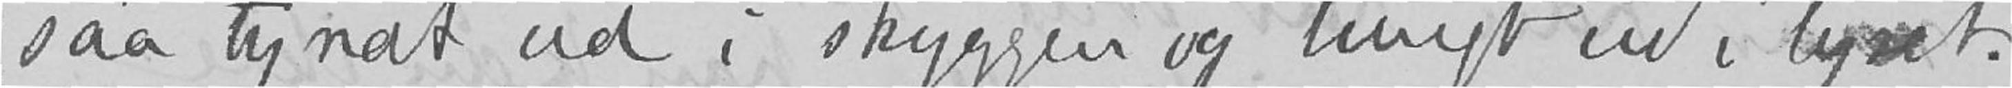saa tyndt ud i skyggen og tungt ud i lyset.
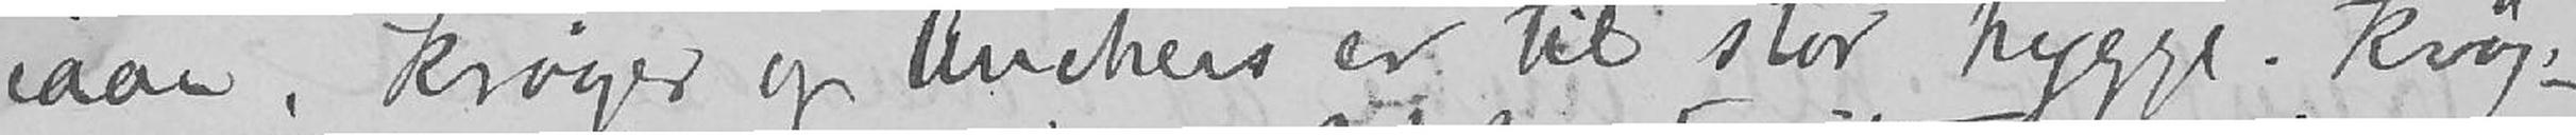iaar, Krøyer og Anchers er til stor hygge. Krøy-
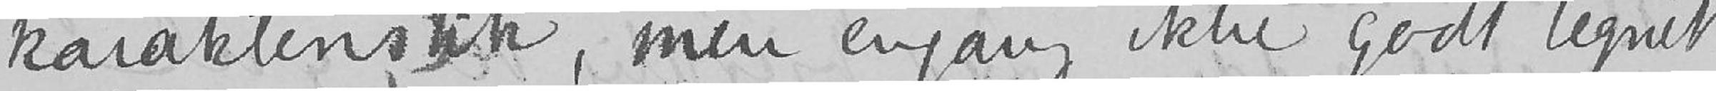karakteristik, men engang ikke godt tegnet
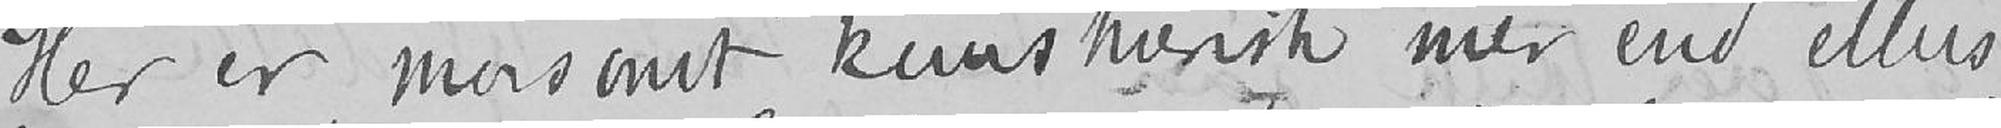Her er morsomt kunstnerisk mer end ellers
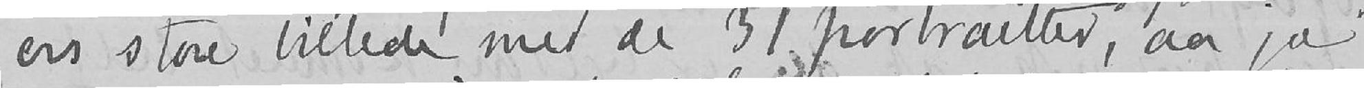ers store billede med 31 portraitter, aa ja
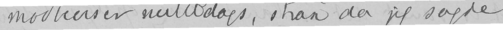modbeviser nutildags, strax da jeg sagde
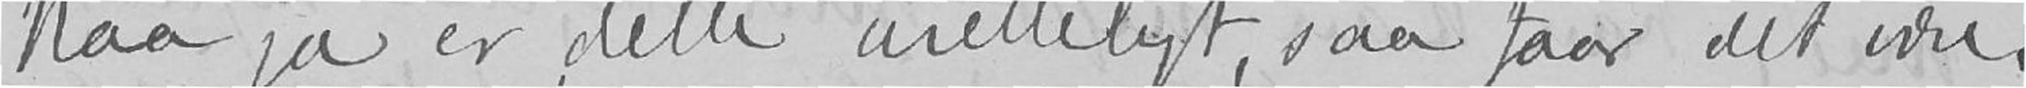Naa ja er dette uretteligt, saa faar det være,
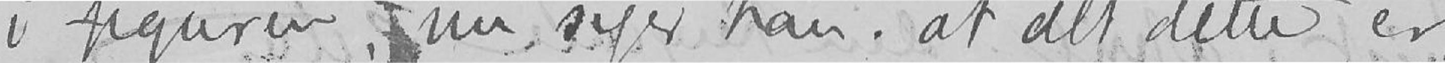i figuren, nu siger han, at alt dette er
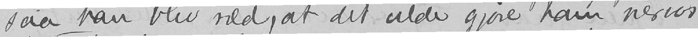saa han blev ræd, at det vilde gjøre ham nervøs
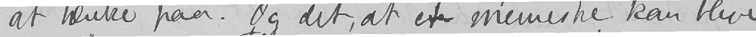at tænke paa. Og det, at et menneske kan blive
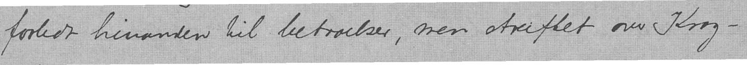forledt hinanden til betroelser, men streiftet over Krag-
